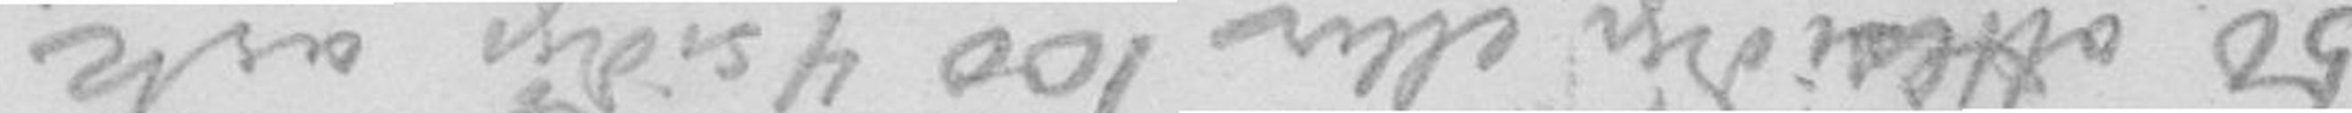50 ottesidige eller 100 4 sidige ark
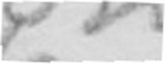Om
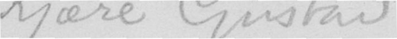Kjære Gustav
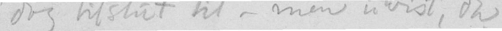dog tilslut til - men uvist, da
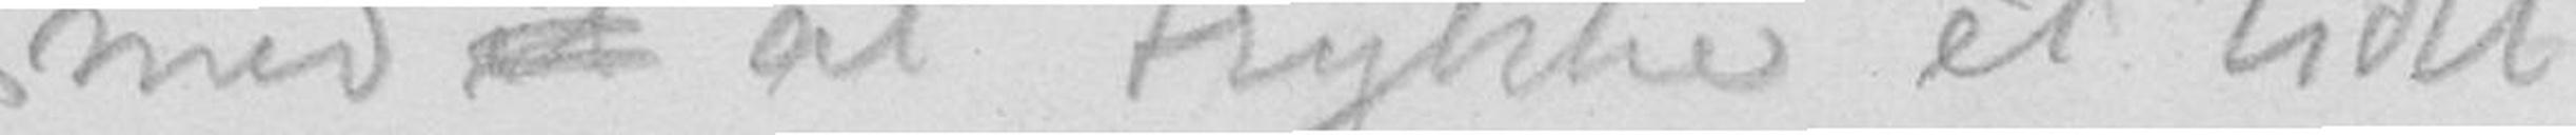med et at trykke et lidet
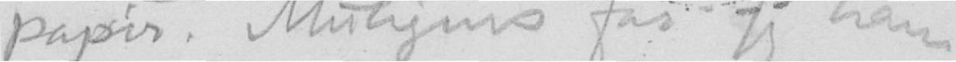papir. Muligens får jeg ham
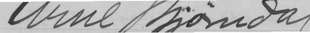Arne Bjørndal
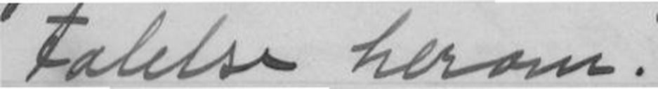talelse herom.
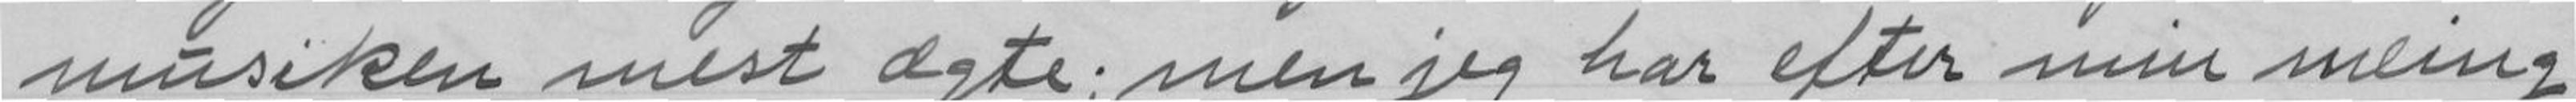musiken mest ægte; men jeg har efter min meing
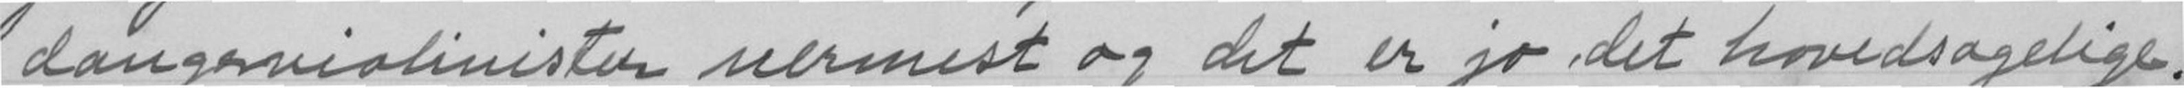dangerviolinister nermest og det er jo det hovedsaglige.
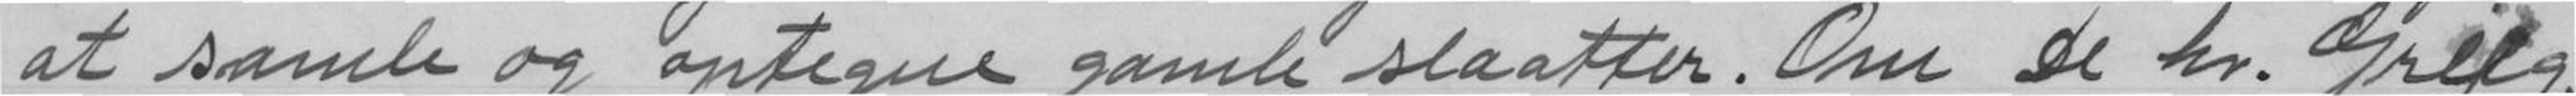at samle og optegne gamle slaatter. Om De hr. Grieg
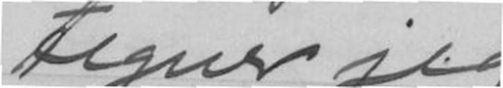tegner jeg
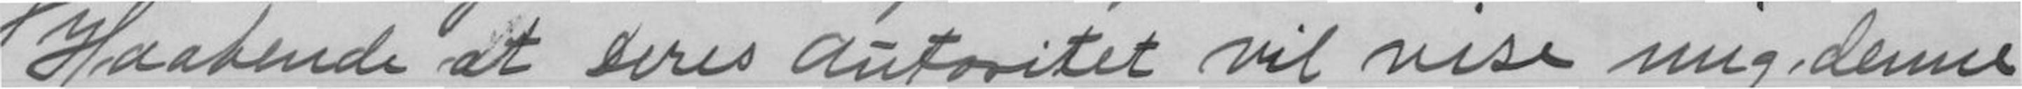Haabende at Deres Autoritet vil vise mig denne
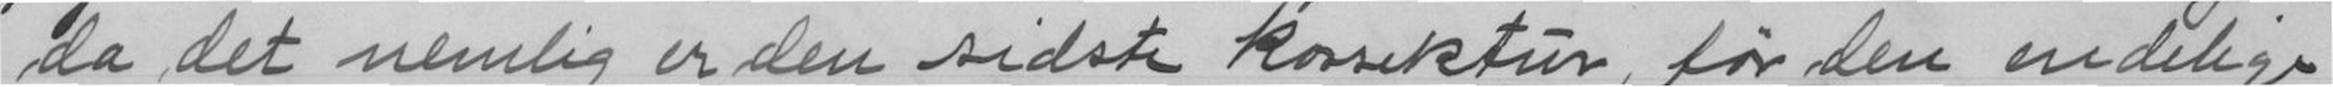da det nemlig er den sidste korrektur, før den endelige
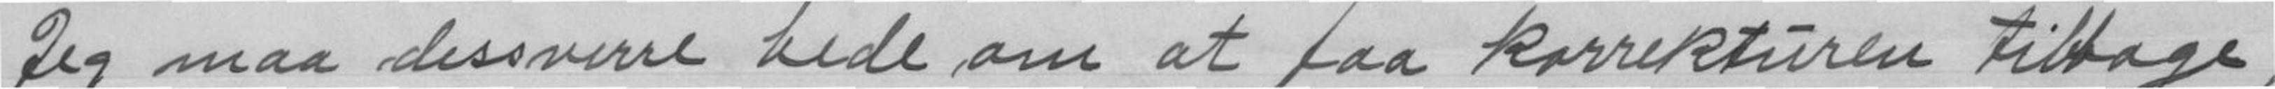Jeg maa desverre bede om at faa korrekturen tilbage,
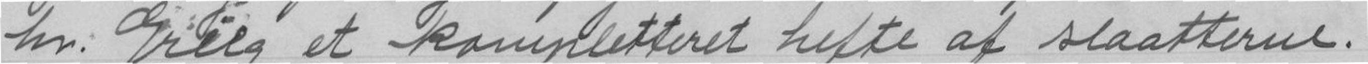hr. Grieg et kompletteret hefte af slaatterne.
